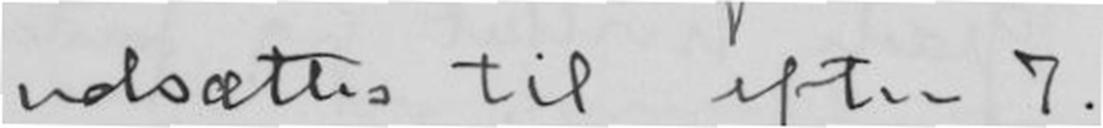udsættes til efter 7.
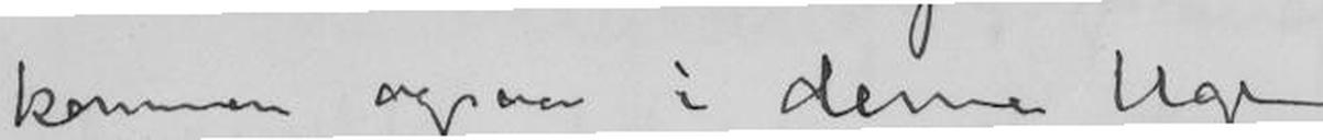kommer ogsaa i dene uge.
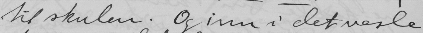til skulen. Og inn i det vesle
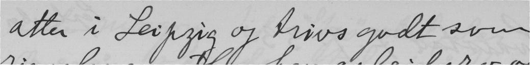atter i Leipzig og trivs godt som
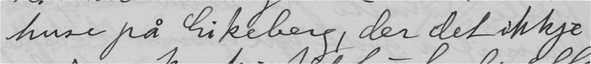huse på Eikeberg, der det ikkje
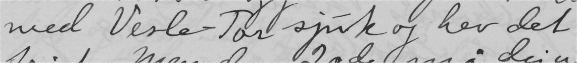med Vesle-Tor sjuk og hev det
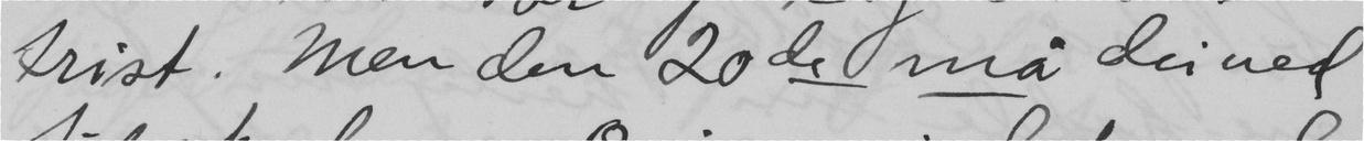trist. Men den 20de må dei ned
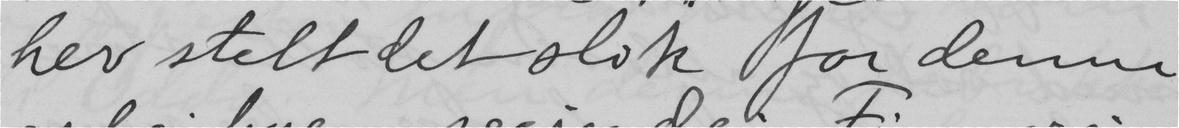hev stelt det slik for denne
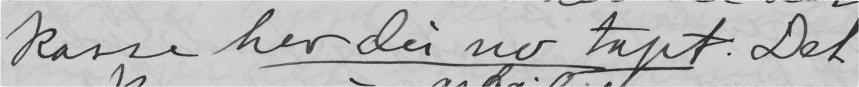kasse hev du no tapt. Det
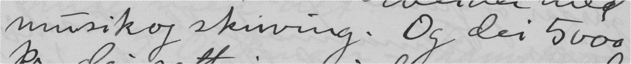musik og skiving. Og dei 5000
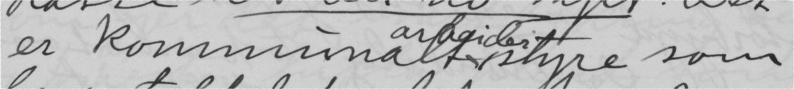er kommunalt arbeiderstyre som
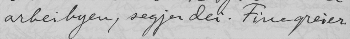arbeibyen, segjer dei. Fine greier.
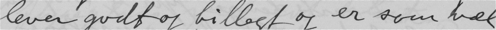lever godt og billegt og er som væl
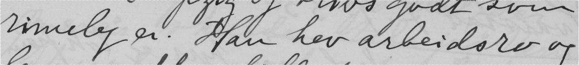rimeleg er. Han hev arbeidsro og
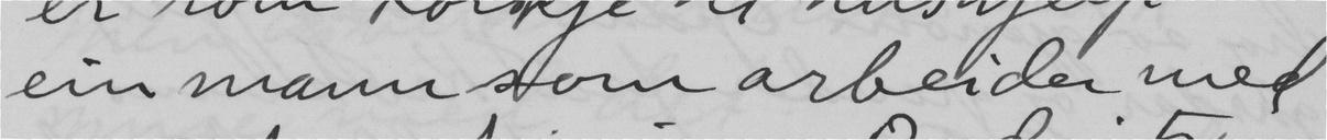ein mann som arbeider med
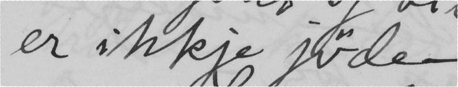er ikkje jöde –
